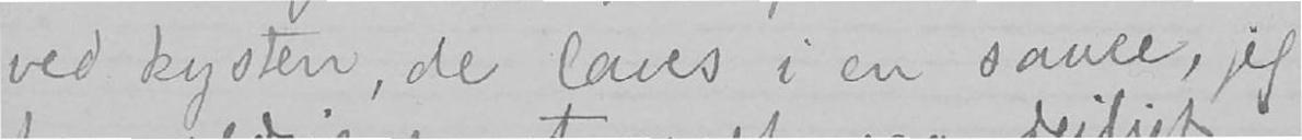ved kysten, de laves i en sauce, jeg
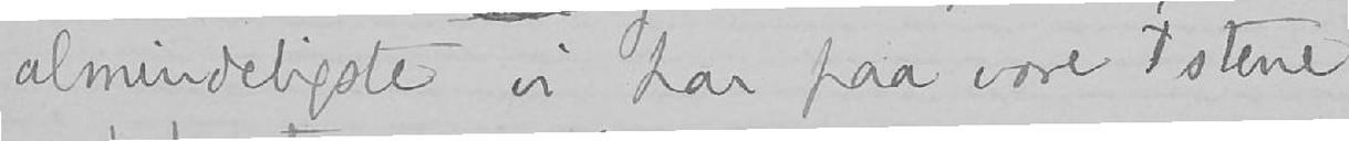almindeligste vi har paa vore t stene
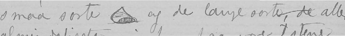smaa sorte og de lange sorte, de aller
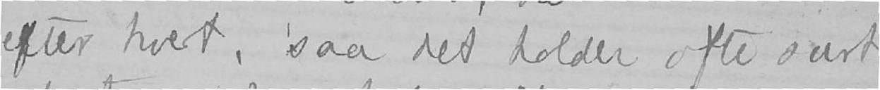efter hvert, saa det holder ofte surt
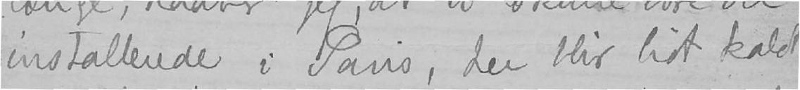installerede i Paris, der blir lidt koldt
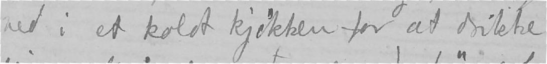ned i et koldt kjøkken for at drikke
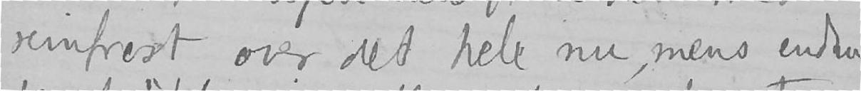rimfrost over det hele nu, mens endnu
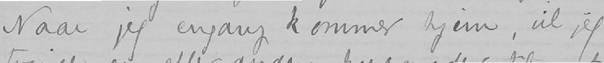Naar jeg engang kommer hjem, vil jeg
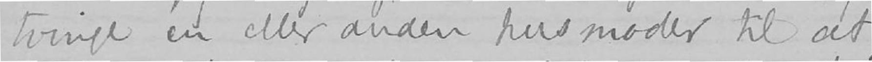tvinge en eller anden husmoder til at
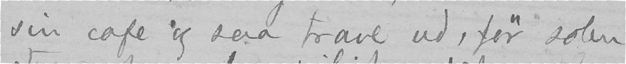sin cafe og saa trave ud, før solen
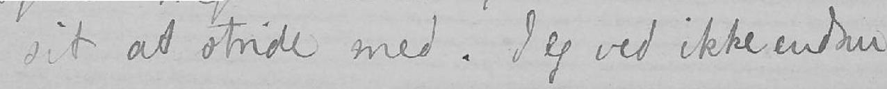sit at stride med. Jeg ved ikke endnu
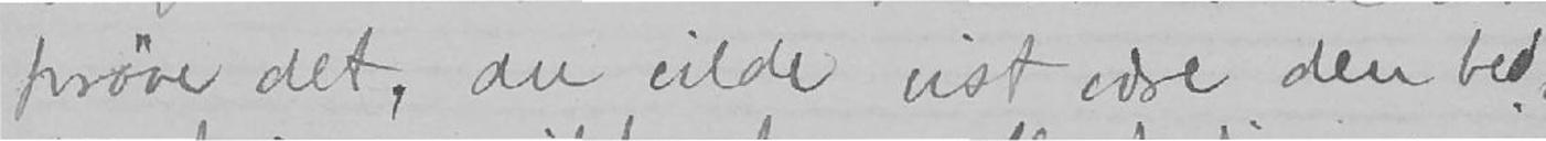prøve det, du vilde vist være den bed-
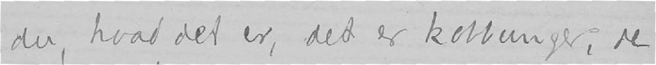du, hvad det er, det er kobbunger, de
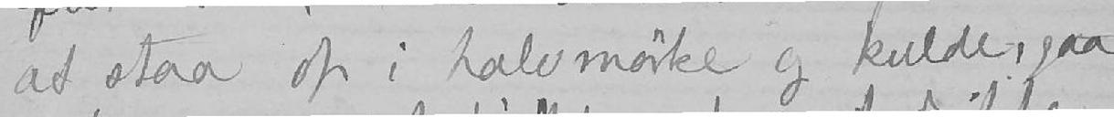at staa op i halvmørke og kulde, gaa
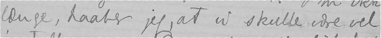længe, haaber jeg, at vi skulle være vel
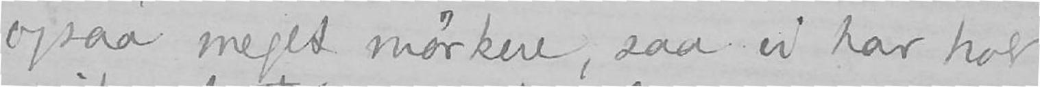ogsaa meget mørkere, saa vi har hat
